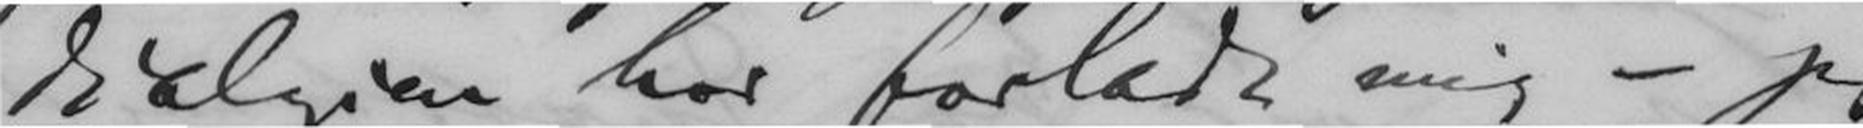dialgien har forladt mig - jeg
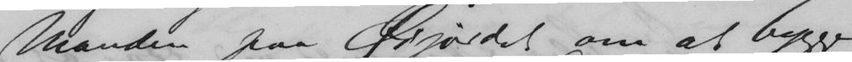Manden paa Øijordet om at bygge
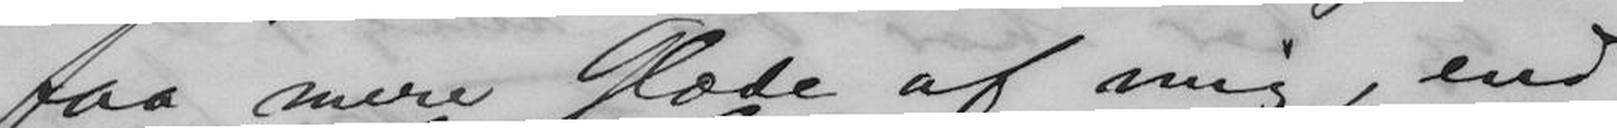faa mere Glæde af mig, end
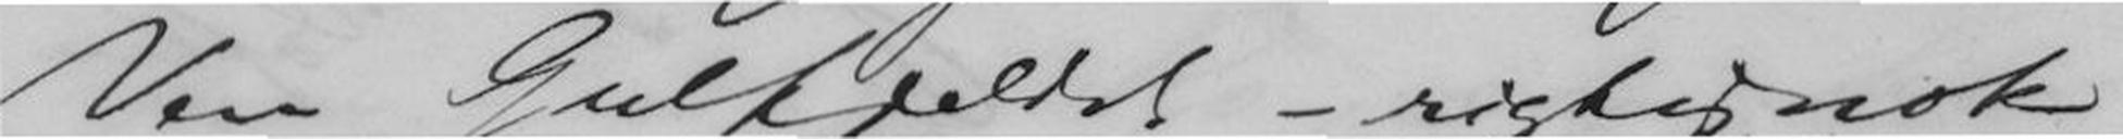Ven Gulfjeldet - rigtignok
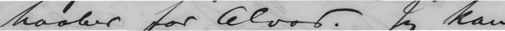haaber for Alvor. Jeg kan
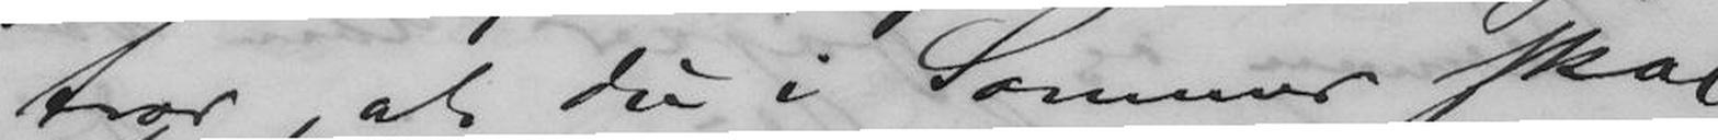tror, at du i Sommer skal
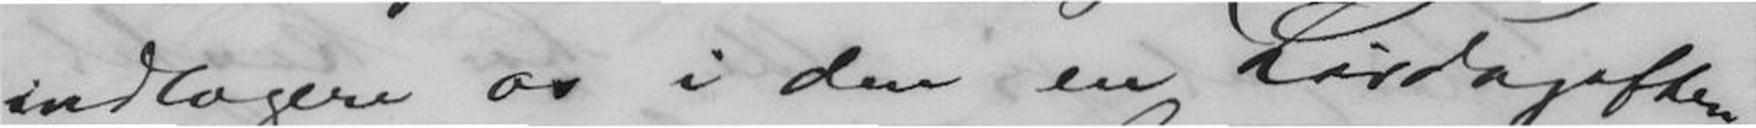indlogere os i den en Lørdagsaften,
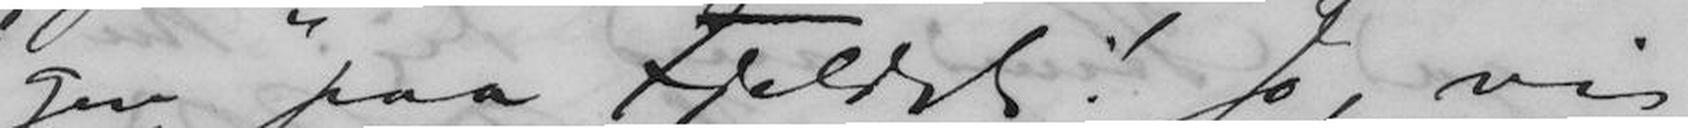gen "paa Fjeldet"! Jo, vi
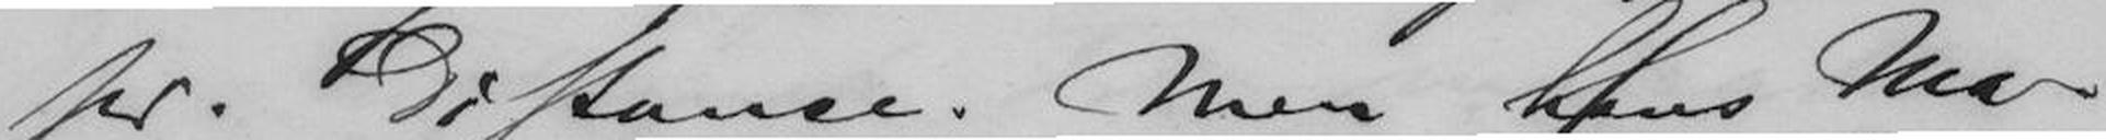pr. Distanse. Men hans Ma-
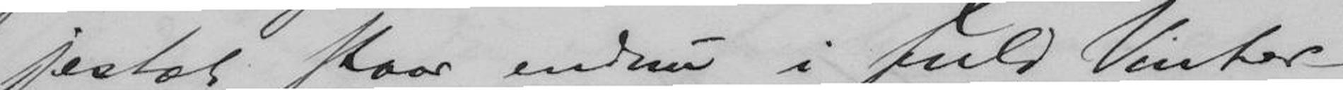jestæt staar endnu i fuld Vinter-
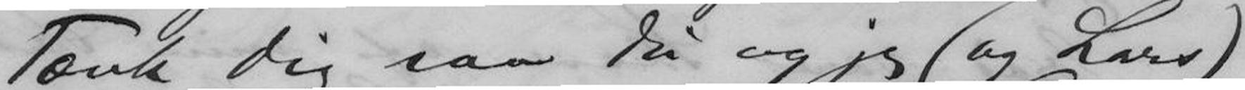Tænk dig saa Du og jeg (og Lars)
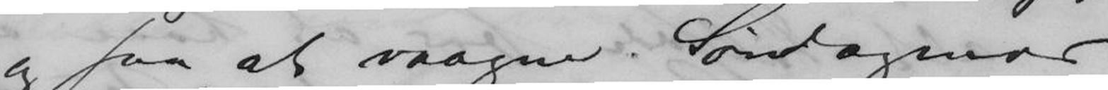og saa at vaagne Søndagsmor-
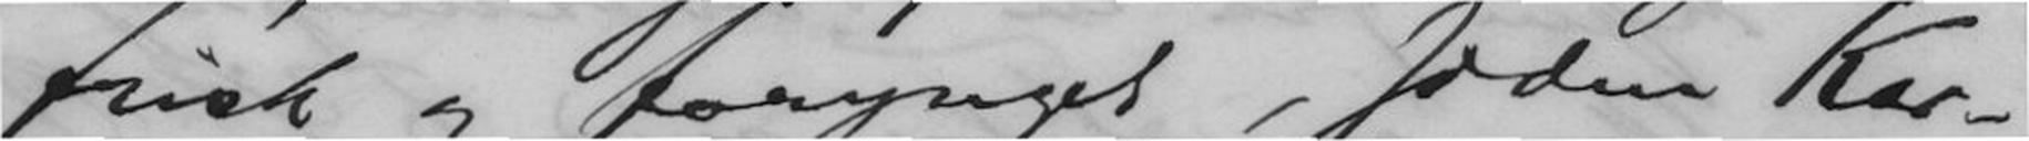frisk og fornyet, siden Kar-
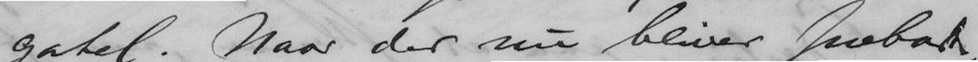gatel. Naar der nu bliver farbart,
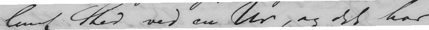lunt Sted ved en Ur, og det har
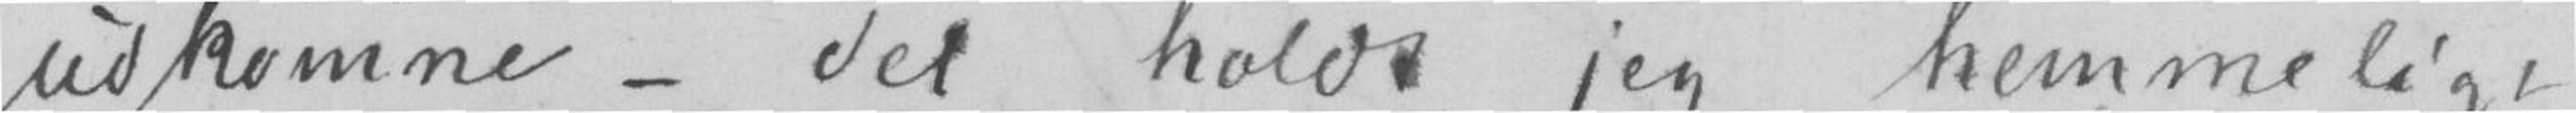udkomne - det holdt jeg hemmelige
